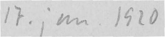17. Jan. 1920
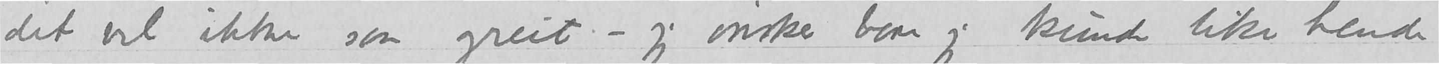det vel ikke saa greit – jeg ønsker bare jeg kunde like hende
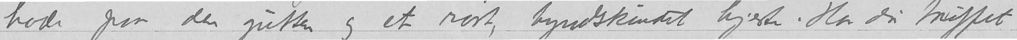hode paa den gutten og et sart, tyndskindet hjerte.  Har du truffet
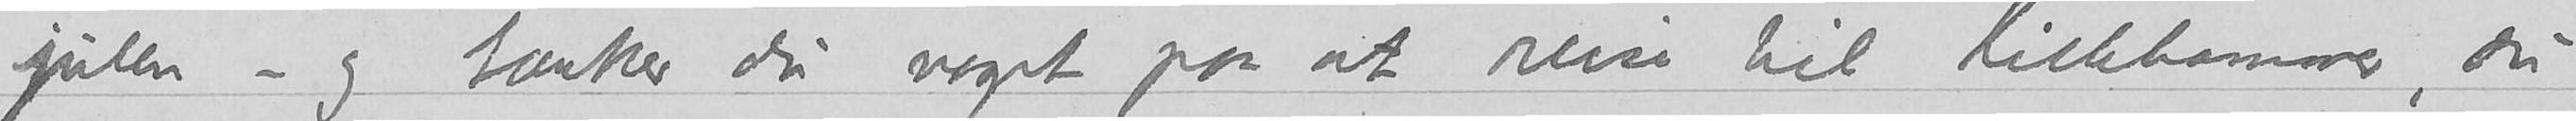julen – og tænker du noget paa at reise til Lillehammer, du
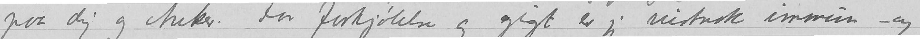paa dig og Anker.  For forkjølelse og gigt er jeg vistnok immun – og
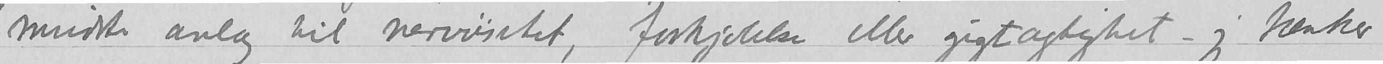mindste anlæg til nervøsitet, forkjølelse eller gigtagtighet – jeg tænker
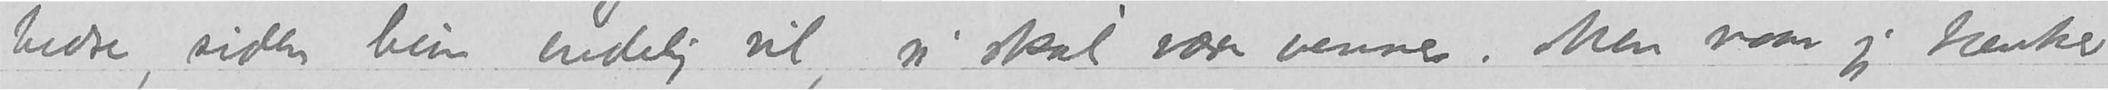bedre, siden hun endelig vil, vi skal være venner.  Men naar jeg tænker
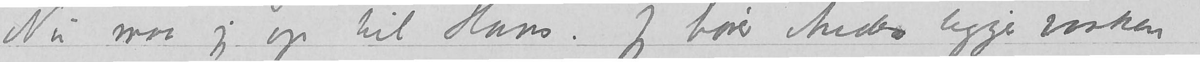Nu maa jeg op til Hans.  Jeg hører Anders ligger vaaken –
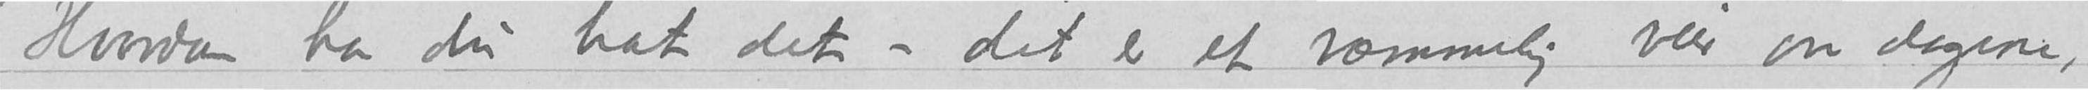Hvordan har du hat det – det er et væmmelig veir om dagene
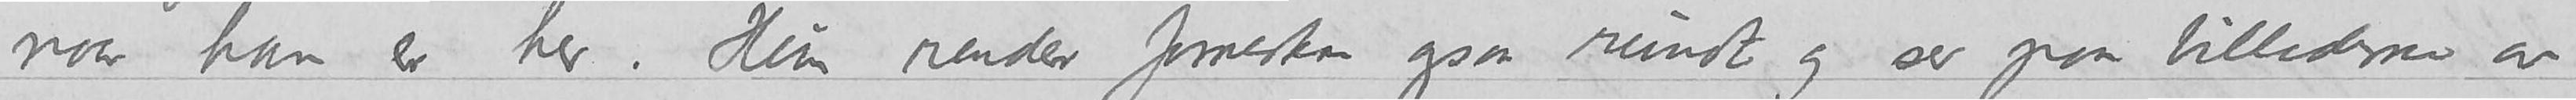naar han er her. Hun render forresten ogsaa rundt og ser paa billederne av
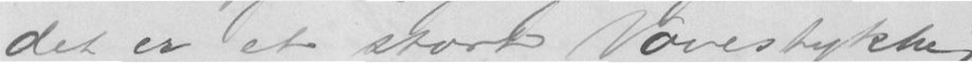det er et stort Vovestykke,
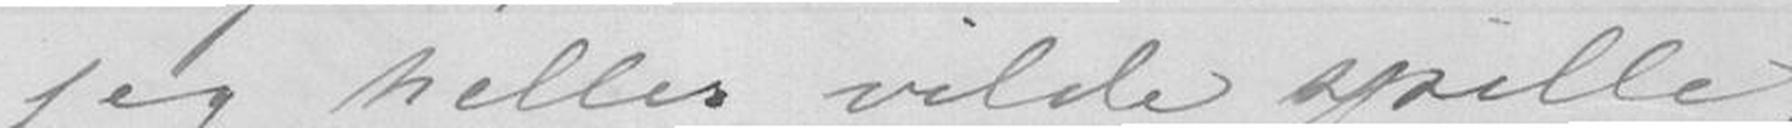jeg heller vilde spille,
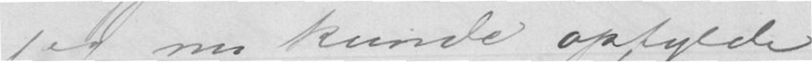jeg nu kunde opfylde
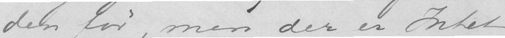den før, men der er Intet
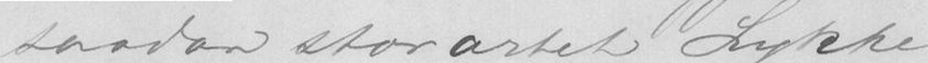saadan storartet Lykke
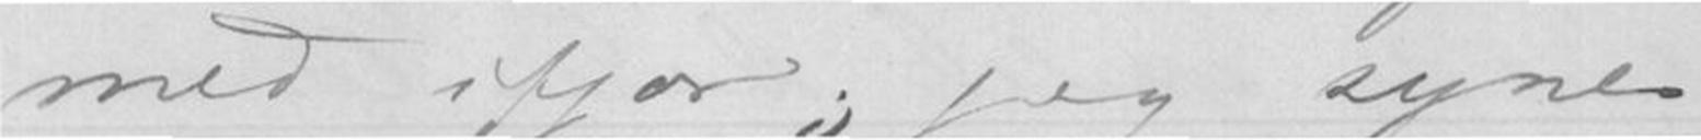med ifjor; jeg synes
